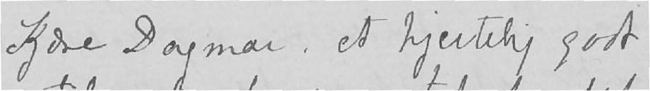Kjære Dagmar, et hjertelig godt
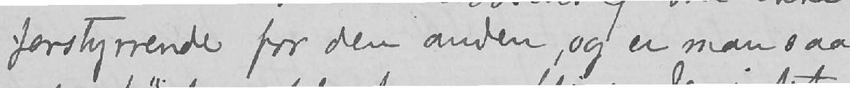forstyrrende for den anden, og er man saa
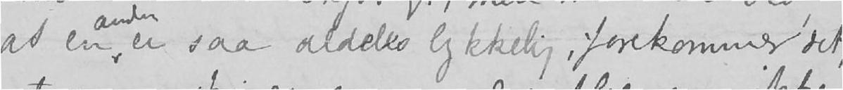at en er saa aldeles lykkelig, forekommer det,
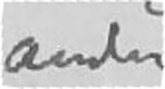anden
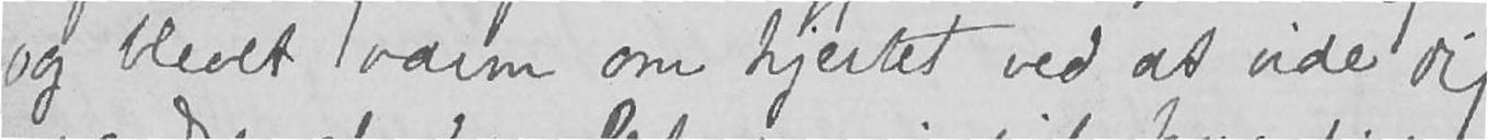og blevet varm om hjertet ved at vide dig
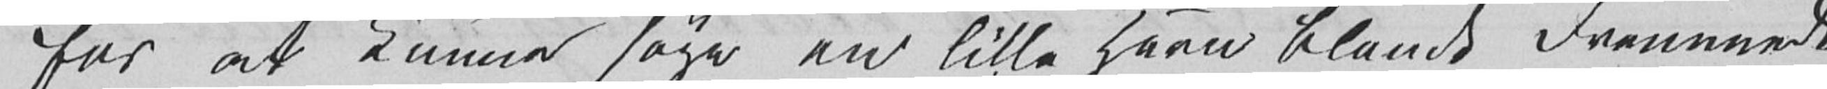for at kunne søge en lille Havn blandt Fremmede
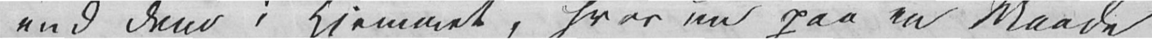end dem i Hjemmet, hvor nu paa en Maade
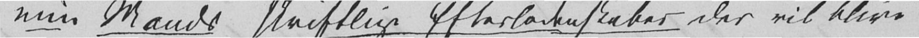min Mands skriftlige Efterladenskaber der vil blive
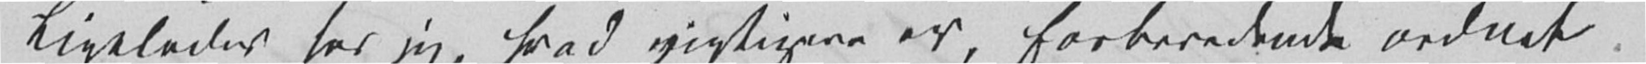Ligeledes har jeg, hvad vigtigere er, forberedende ordnet
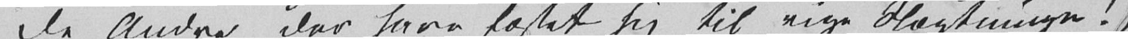de Andre der have fæstet sig til rige Slægtninge!)
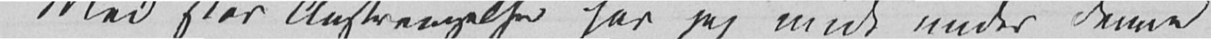Med stor Anstrengelse har jeg midt under denne
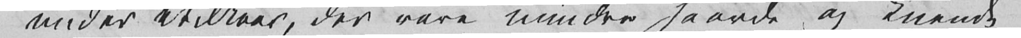under Vilkaar, der vare mindre haarde og kuende
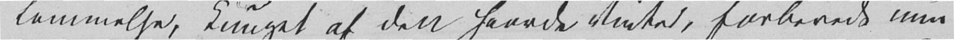Lammelse, knuget af den haarde Vinter, forberedt min
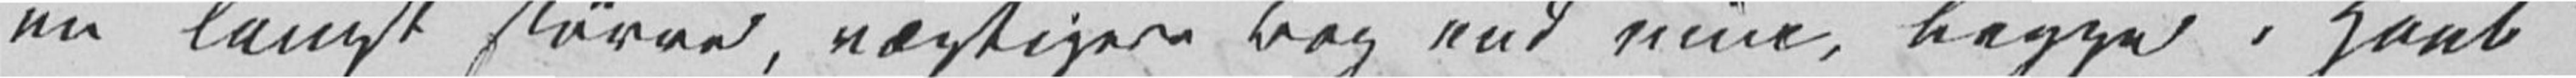en langt større, vægtigere Bog end min, begge i Haab
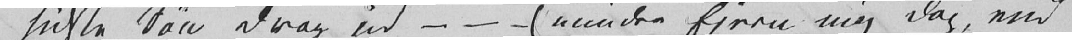sidste Søn drog ud – – – (mindre fjern mig dog, end
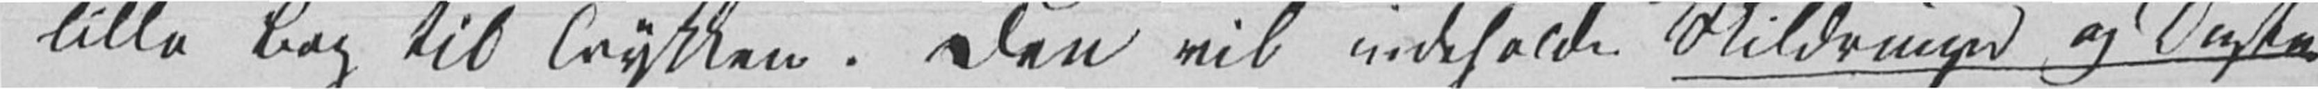lille Bog til Trykken. Den vil indeholde Skildringer og Digte.
